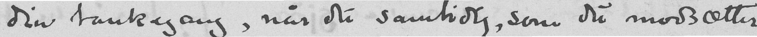din tankegang, når du samtidig, som du modsætter
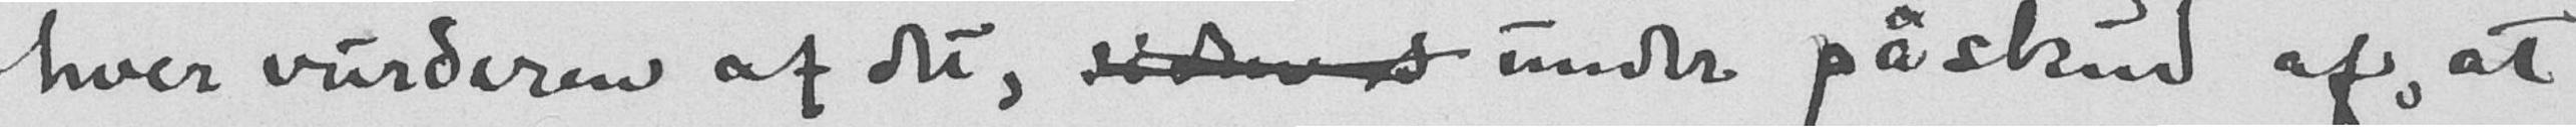hver vurderen af det, siden d under påskud af, at
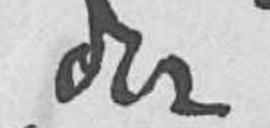der
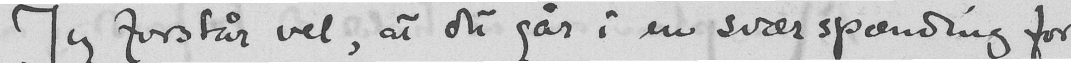Jeg forstår vel, at du går i en svær spænding for
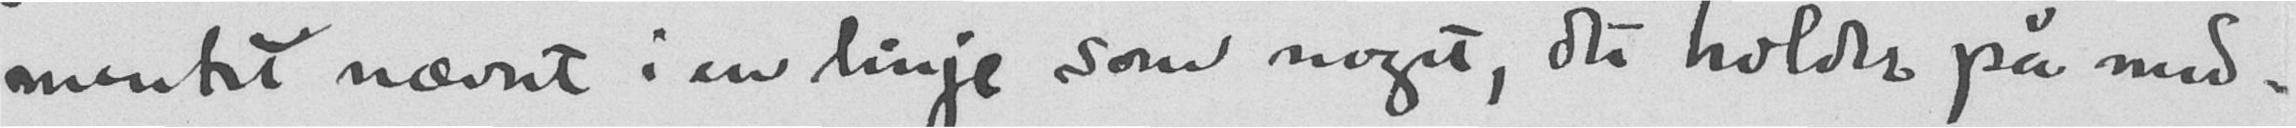mentet nævnt i en linje som noget, du holder på med.
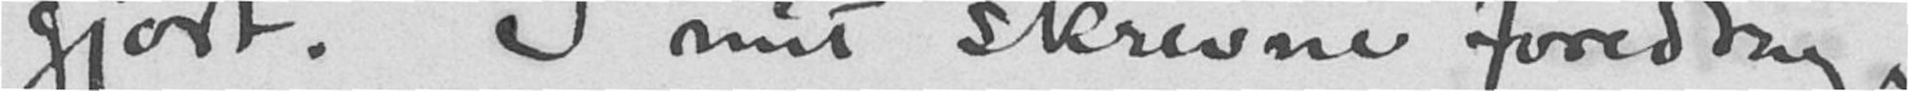gjort. I mit skrevne foredrag
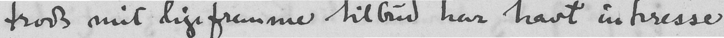trods mit ligefremme tilbud har havt interesse
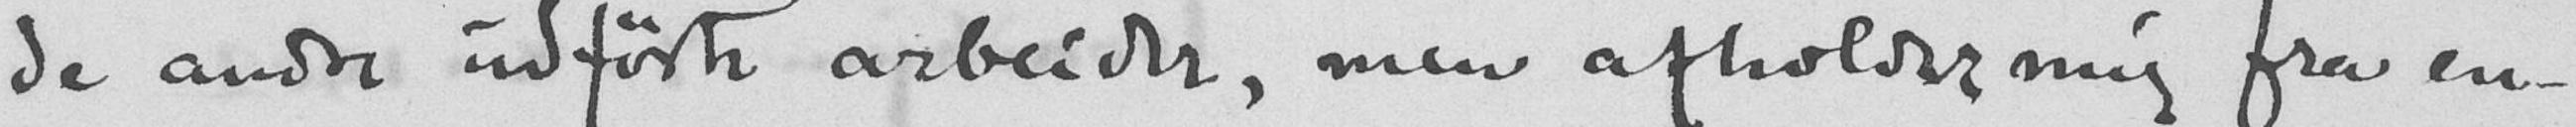de andre udførte arbeider, men afholder mig fra en-
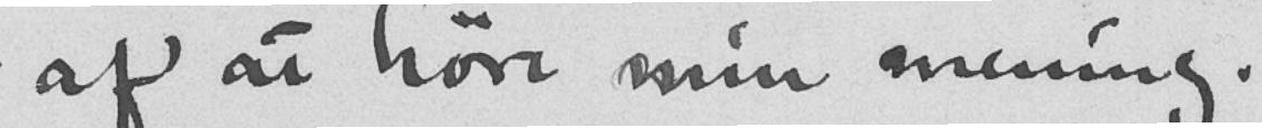af at høre min mening.
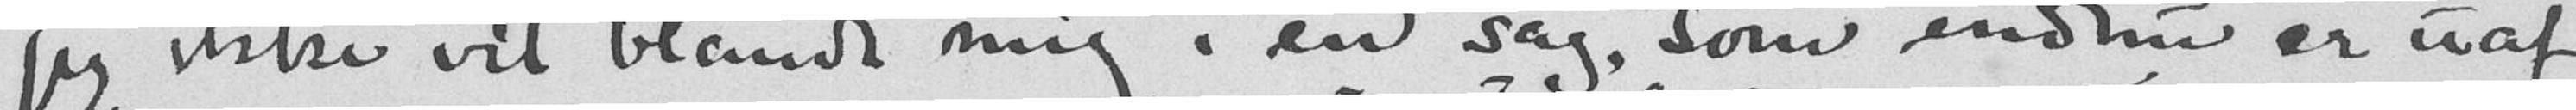jeg ikke vil blande mig i en sag, som endnu er uaf-
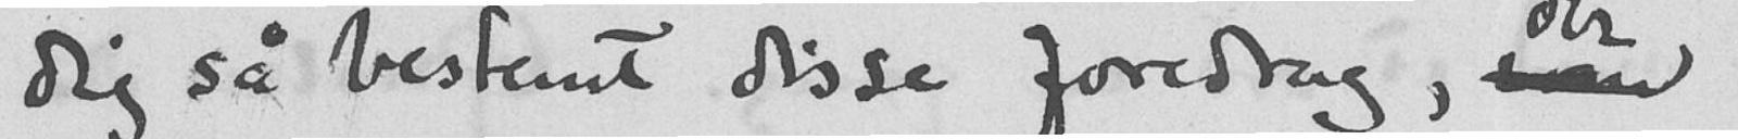dig så bestemt disse foredrag, som
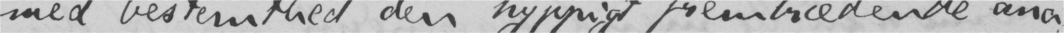med bestemthed den hyppigt fremtrædende ana-
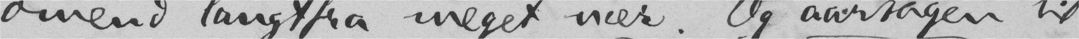omend langtfra meget nær. Og aarsagen til
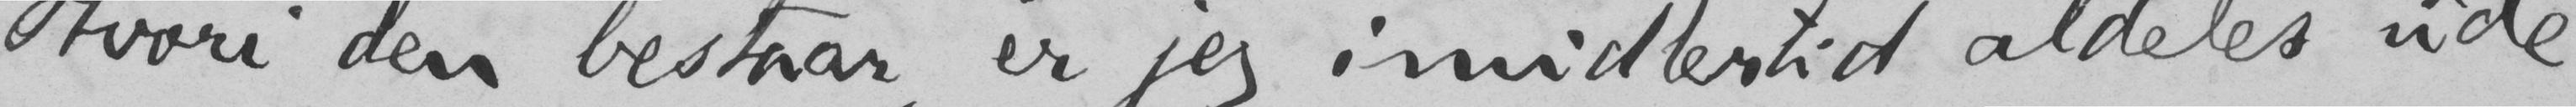Hvori den bestaar, er jeg imidlertid aldeles ude
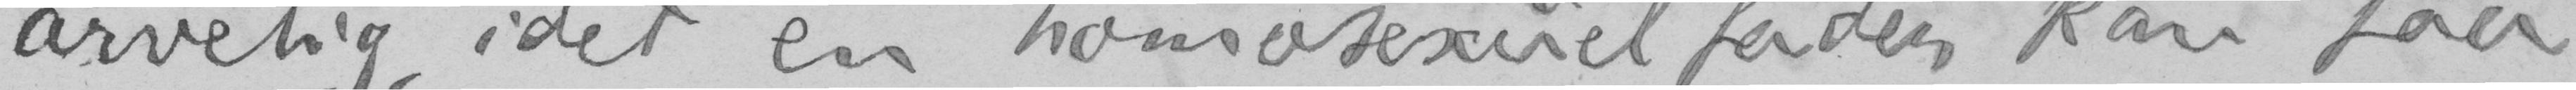arvelig, idet en homosexuel fader kan faa
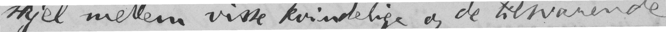skjel mellem visse kvindelige og de tilsvarende
